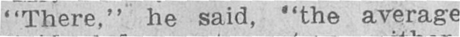"There," he said, "the average
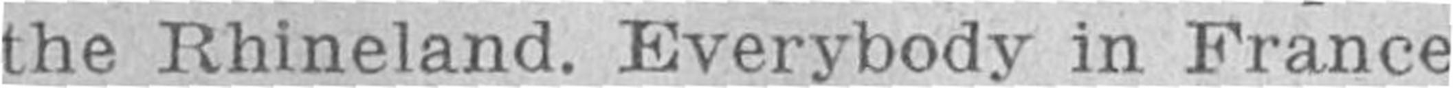the Rhineland. Everybody in France
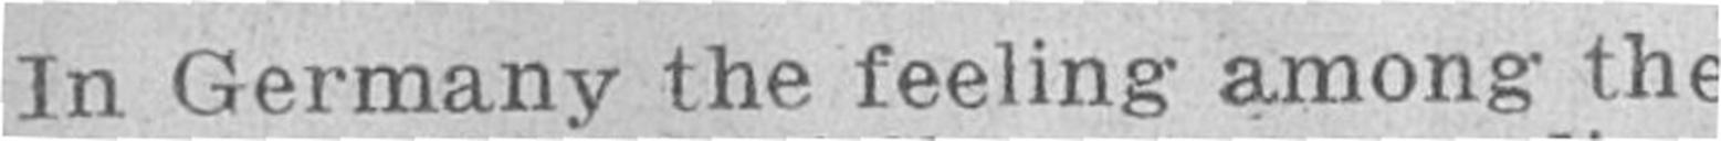In Germany the feeling among the
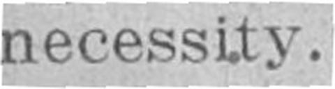necessity.
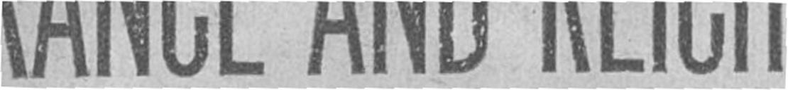FRANCE AND REICH
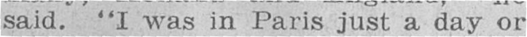said. "I was in Paris just a day or
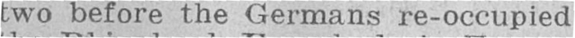two before the Germans re-occupied
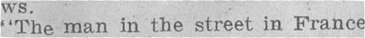"The man in the street in France
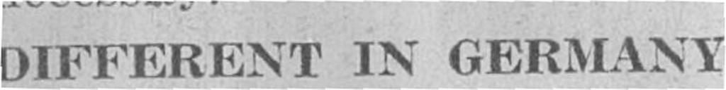DIFFERENT IN GERMANY
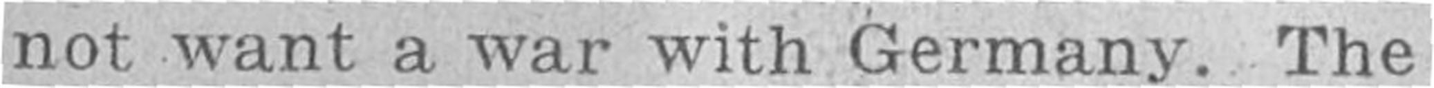not want a war with Germany. The
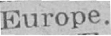Europe.
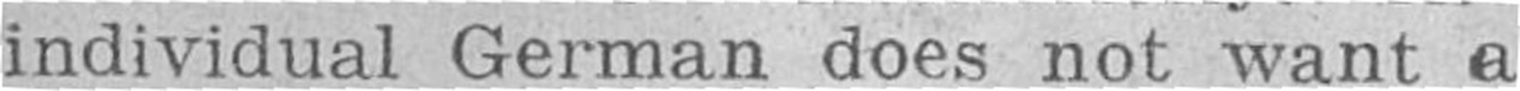individual German does not want a
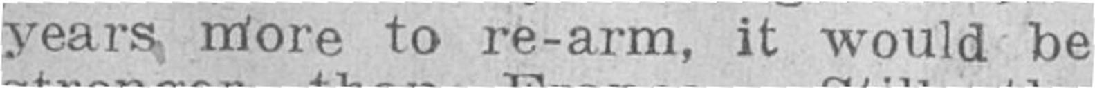years more to re-arm, it would be
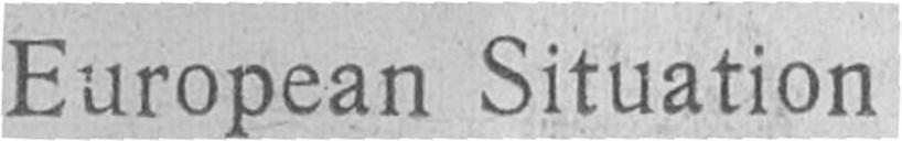European Situation
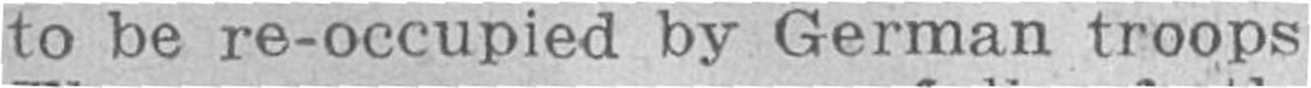to be re-occupied by German troops
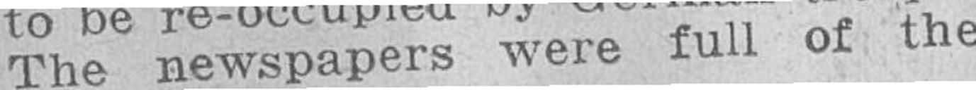The newspapers were full of the
